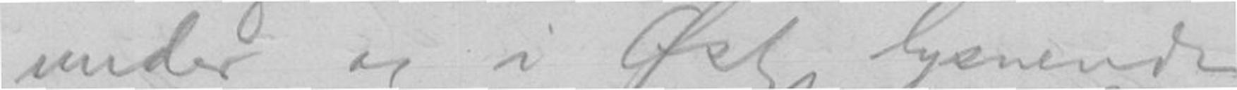under og i Øst lysnende
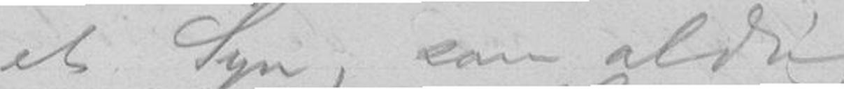- - et Syn, som aldrig
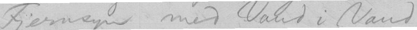Fjernsyn med Vand i Vand
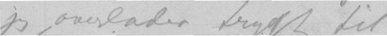- - - - jeg overlader trygt til
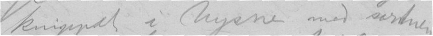knippet i Nysne med sortnen-
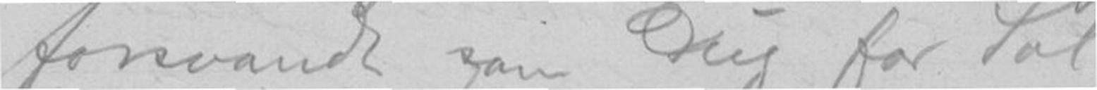forsvandt som Dug for Sol, og
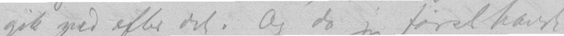gik ned efter det. Og da jeg først havde
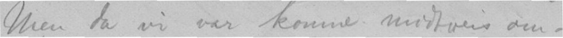Men da vi var komne midtveis om-
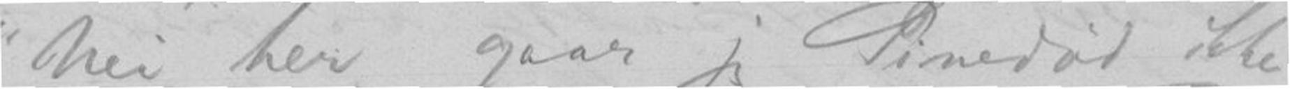"Nei her gaar jeg Pinadød ikke
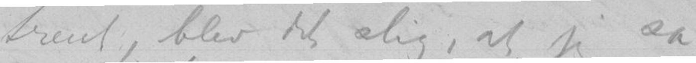trent, blev det slig, at jeg sa:
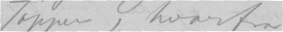Toppen, hvorfra - - -
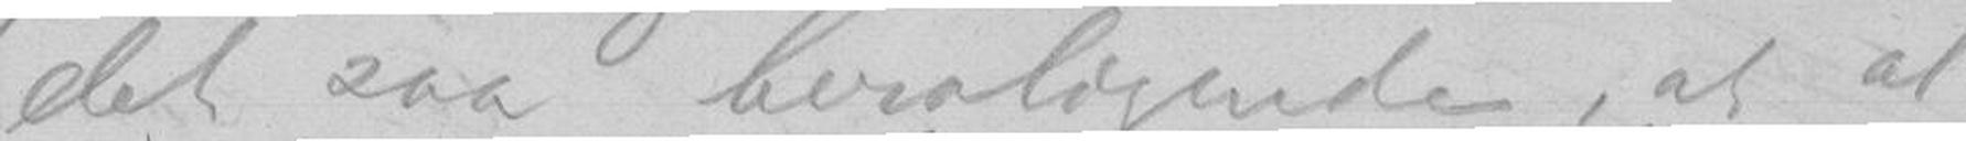det saa beroligende, at al
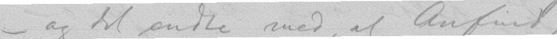- og det endte med, at Anfind
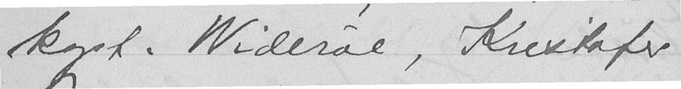kapt. Widerøe, Kristofer
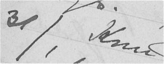31/1 Kom
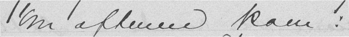Om aftenen kom:
